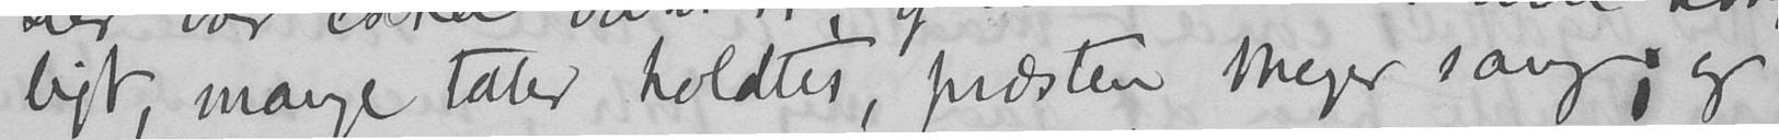ligt, mange taler holdtes, præsten Meyer sang i og
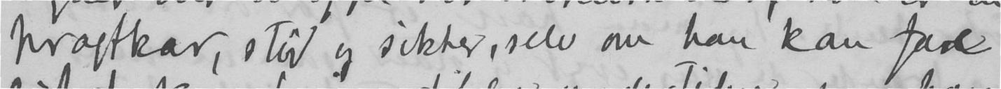pragtkar, stor og sikker, selv om han kan fare
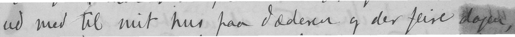ud med til mit hus paa Jæderen og der feire dagen,
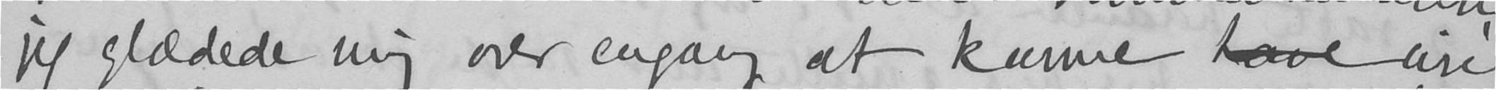jeg glædede mig over engang at kunne have vie
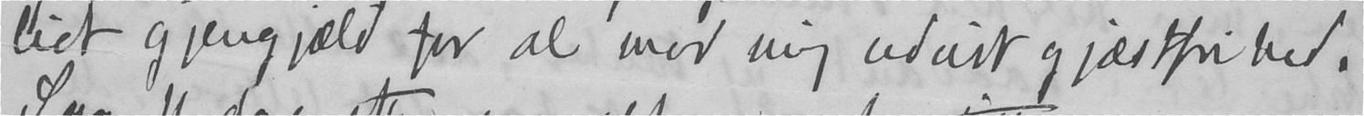lidt gjengjæld for al mod mig udvist gjæstfrihed.
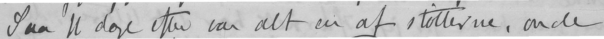Saa 11 dage efter var alt en af støtterne, oncle
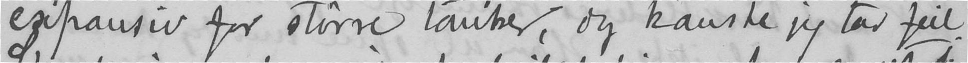expansiv for større tanker, og kanske jeg tar feil.
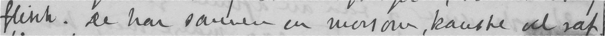flink. De har sammen en morsom, kanske vel raf-
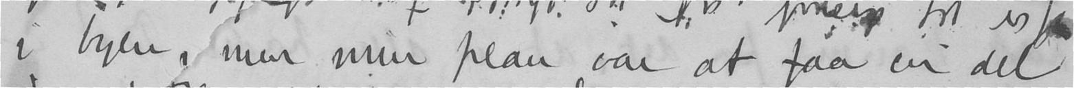i byen, men min plan var at faa en del
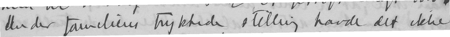Under familiens trykkede stilling havde det ikke
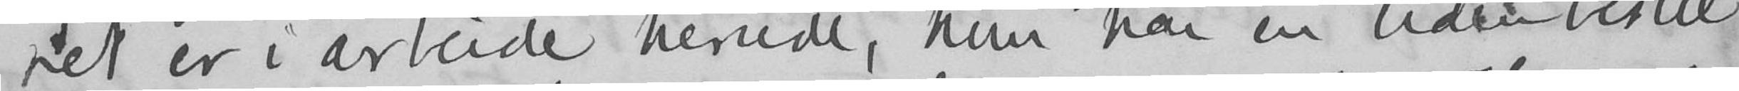riet er i arbeide herude, hun har en liden bestil-
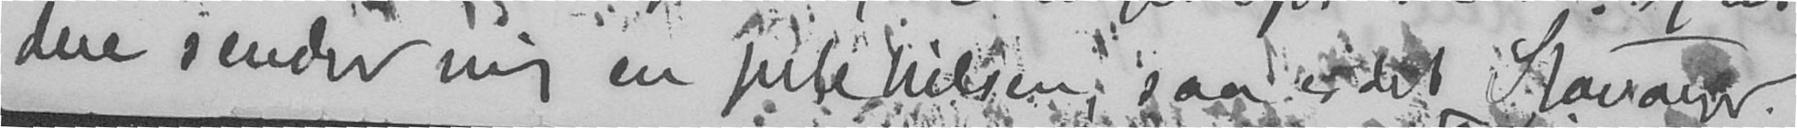dere sender mig en julehilsen, saa er det Stavanger.
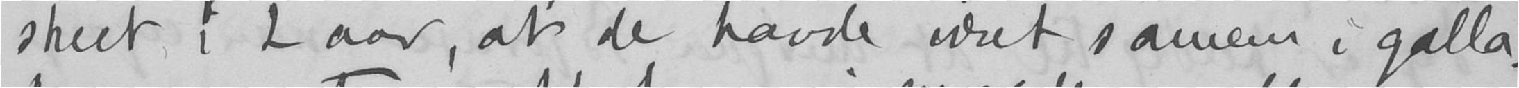skeet i 2 aar, at de havde været sammen i galla.
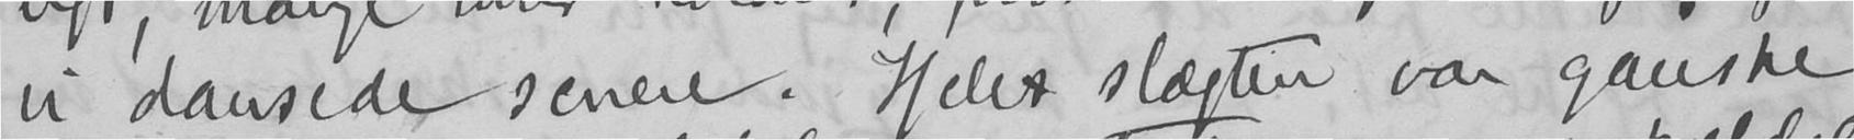vi dansede senere. Heles slægten var ganske
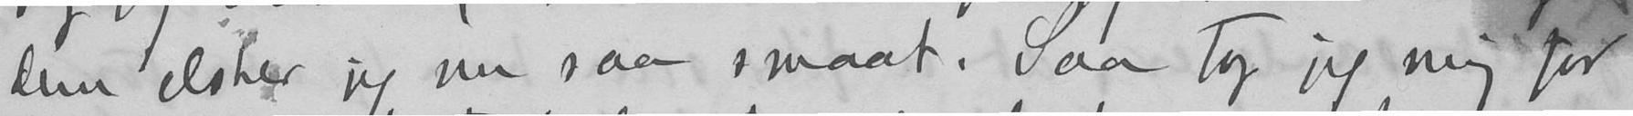dem elsker jeg nu saa smaat. Saa tog jeg mig for
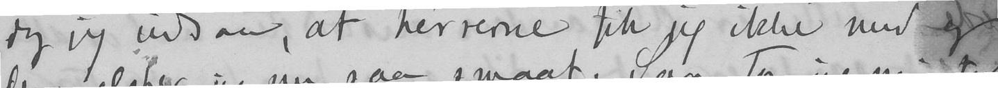dog jeg indsaa, at herrerne fik jeg ikke med og
